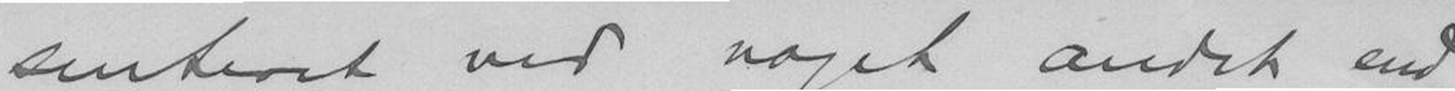senteret ved noget andet end
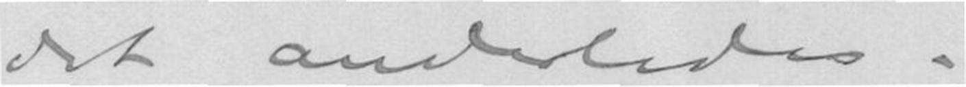det anderledes.
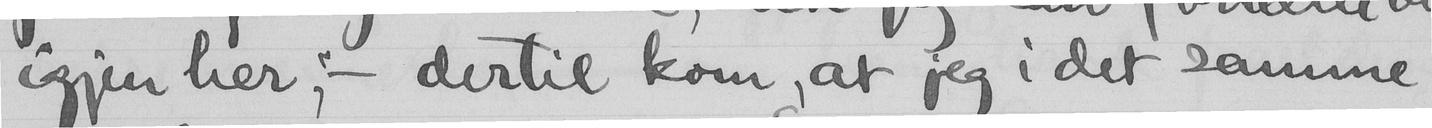igjen her; - dertil kom, at jeg i det samme
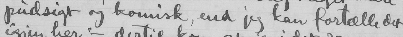pudsigt og komisk, end jeg kan fortælle det
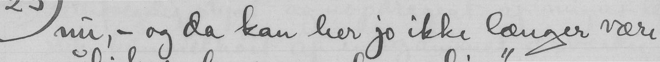nu, - og da kan her jo ikke længer være
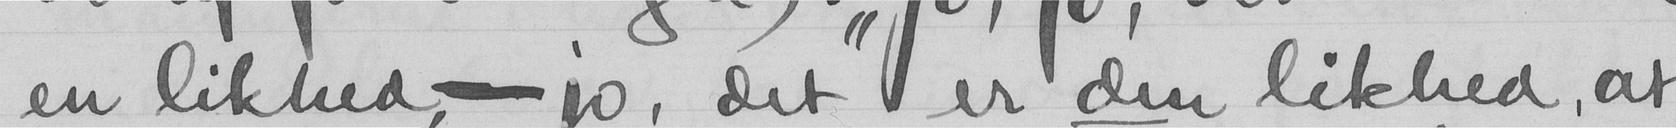en likhed,- jo, det ver den likhed, at
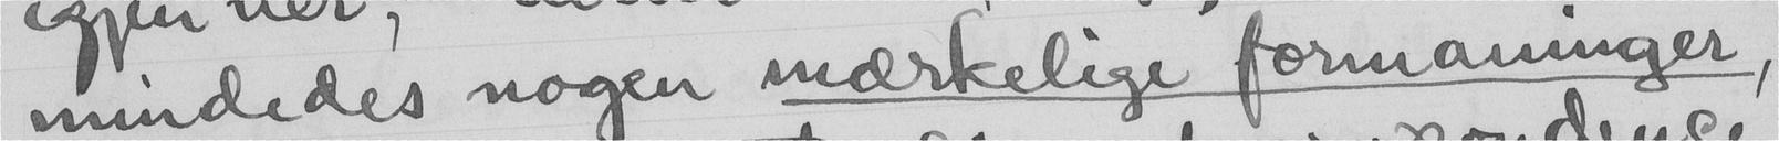mindedes nogen mærkelige formaninger,
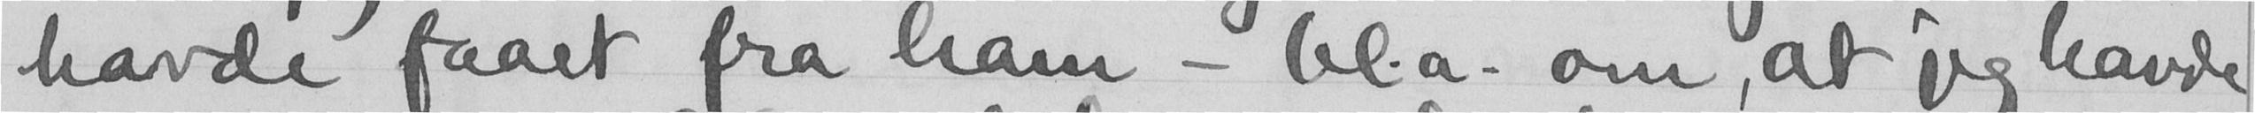havde faaet fra ham - bla. om, at jeg havde
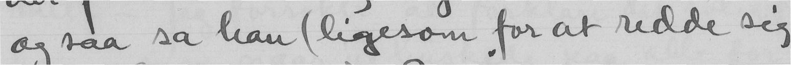og saa sa han (ligesom for at redde sig
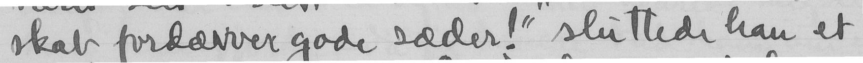skab fordærver gode sæder!" sluttede han et
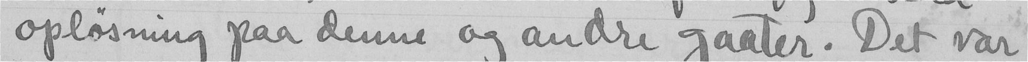opløsning paa denne og andre gaater. Det var
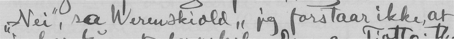"Nei", sa Werenskiold," jeg forstaar ikke, at
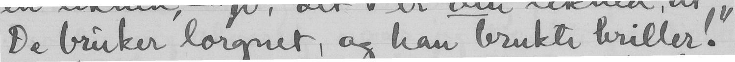De bruker lorgnet, og han brukte briller!"
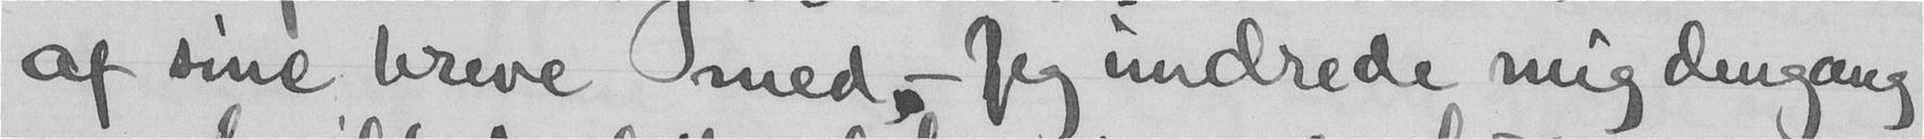af sine breve med.- Jeg undrede mig dengang
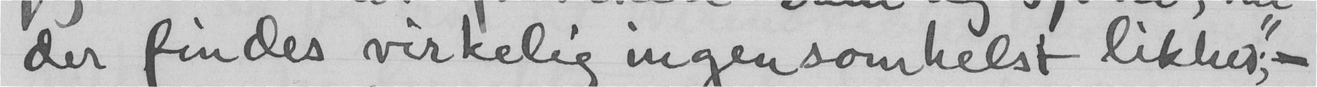der findes virkelig ingensomhelst likhed,-
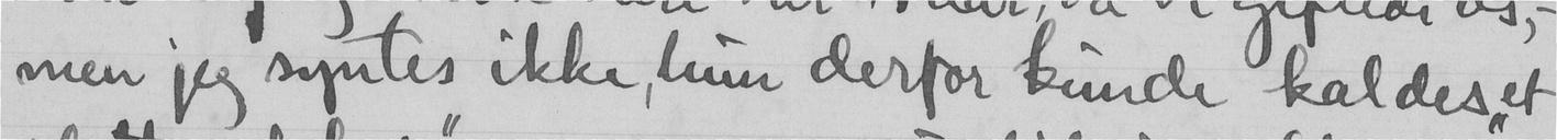men jeg syntes ikke, hun derfor kunde kaldes "et
